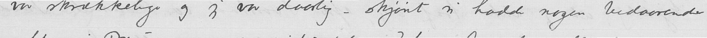var skrækkelige og jeg var daarlig - skjønt vi hadde nogen bedaarende
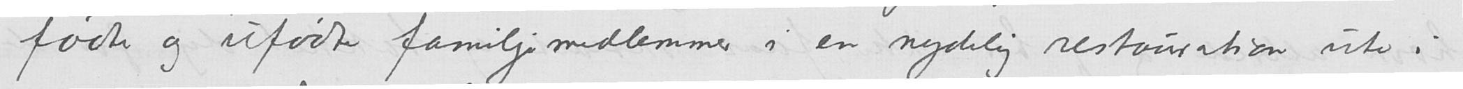fødte og ufødte familjemedlemmer i en nydelig restauration ute i
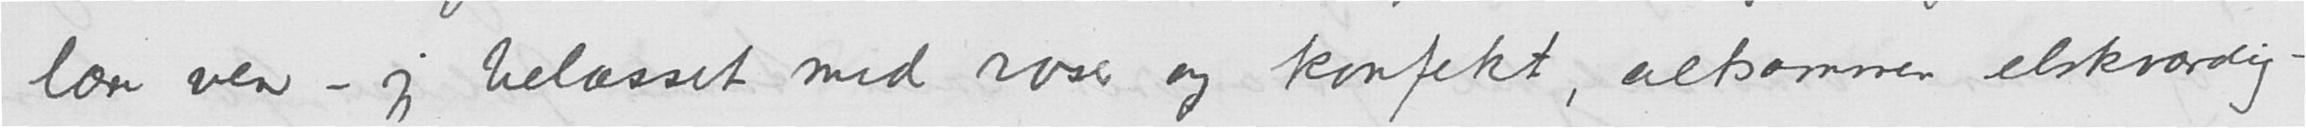lære ven - jeg belæsset med roser og konfekt, altsammen elskværdig-
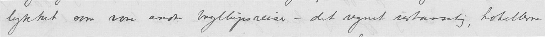lykket som vore andre bryllupsreiser - det regnet ustanselig, hotellerne
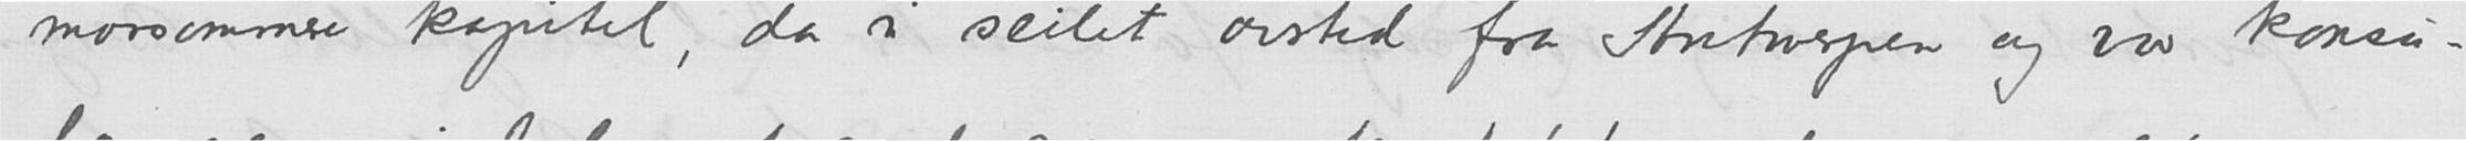morsommere kapitel, da vi seilet avsted fra Antwerpen og vor konsu-
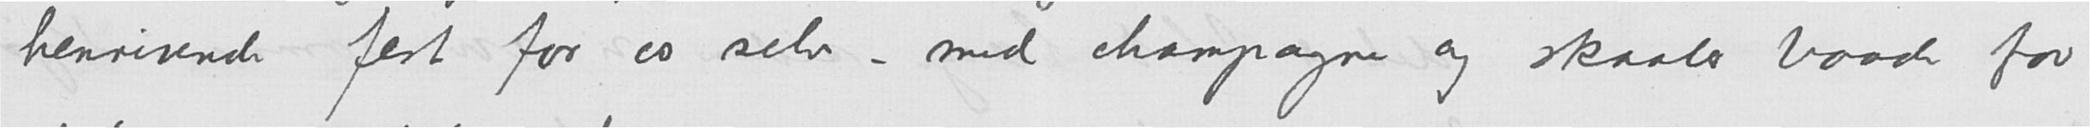henrivende fest for os selv - med champagne og skaaler baade for
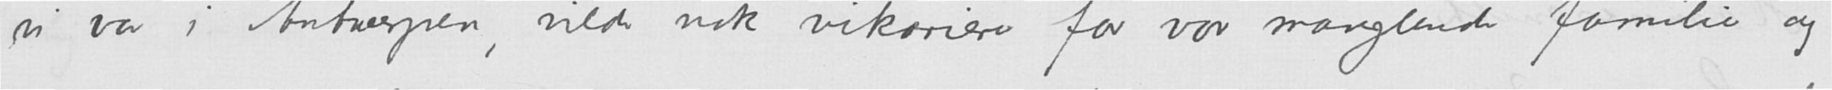vi var i Antwerpen, vilde nok vikariere for vor manglende familie og
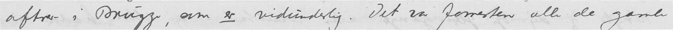aftener i Brügge, som er vidunderlig. Det var forresten alle de gamle
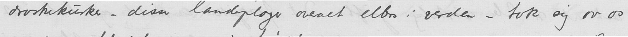droskekusker - disse landeplage overalt ellers i verden - tok sig av os
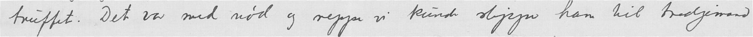truffet, Det var med nød og neppe vi kunde slippe ham til tredjemand
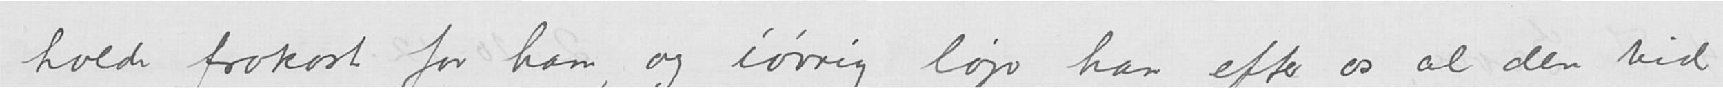holde frokost for ham, og iøvrig løp han efter os al den tid
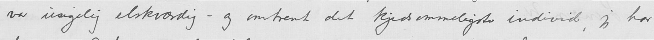var usigelig elskværdig - og omtrent det kjedsommeligste individ, jeg har
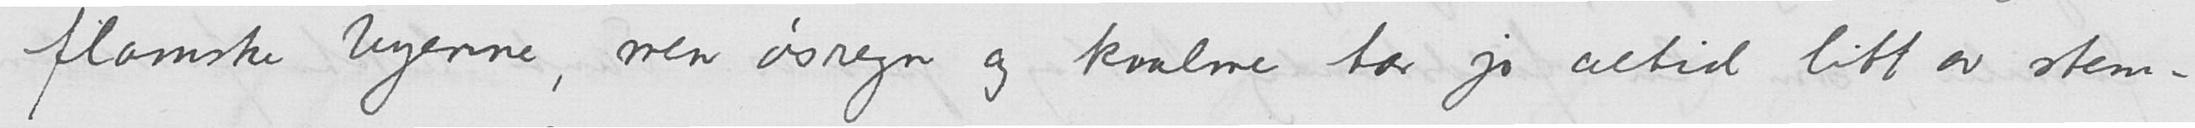flamske byerne, men øsregn og kvalme tar jo altid litt av stem-
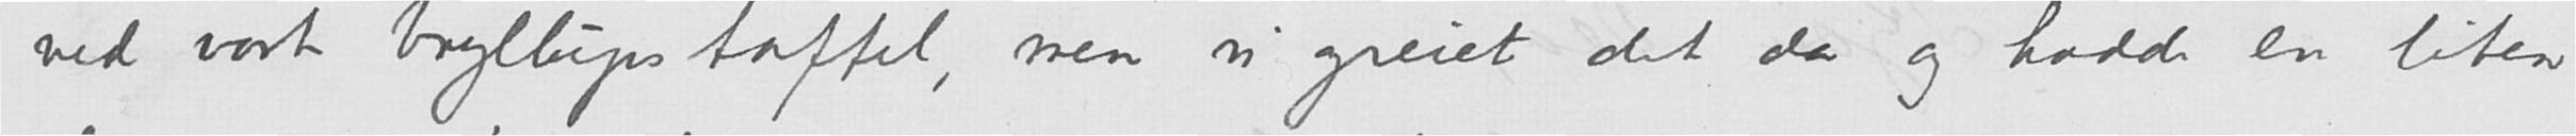ved vort bryllupstaffel, men vi greiet det da og hadde en liten
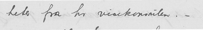heter fra hr. vicekonsulen. -
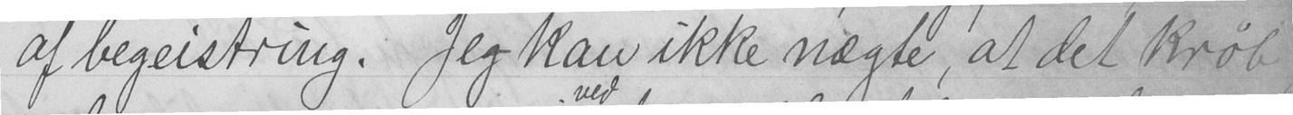af begeistring. Jeg kan ikke nægte, at det krøb
